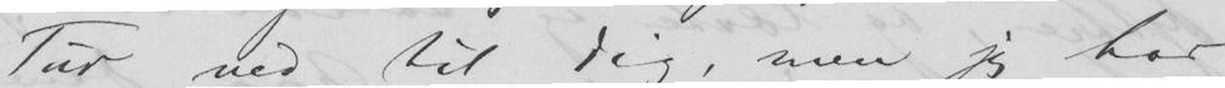Tur ned til Dig, men jeg har
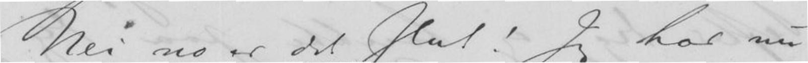Nei no er det slut! Jeg har nu
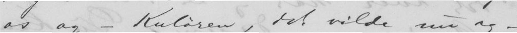os og - Kuløren, det vilde nu og-
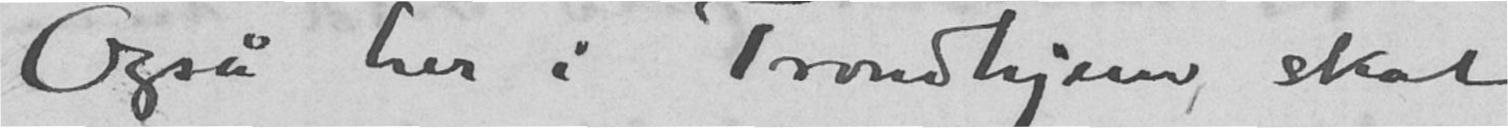Også her i Trondhjem skal
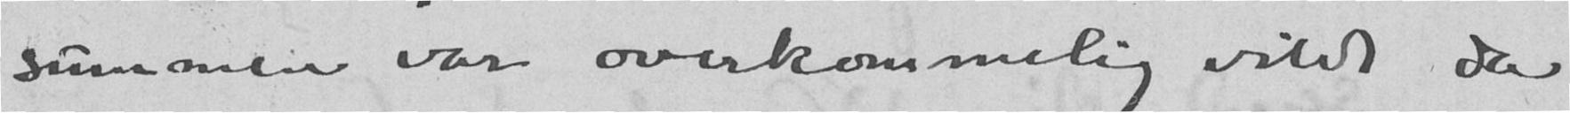summen var overkommelig vilde da
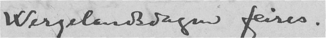Wergelandsdagen feires.
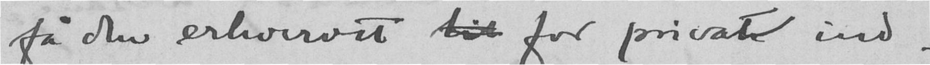få den erhvervet til for privat ind-
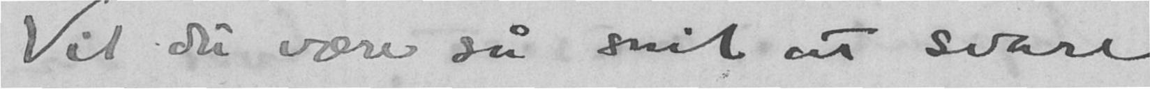Vil du være så snil at svare
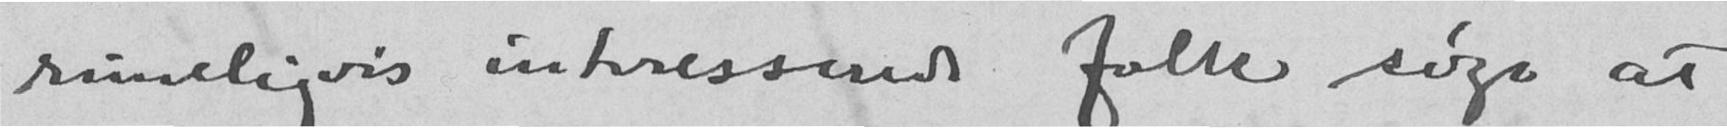rimeligvis interesserede folk søge at
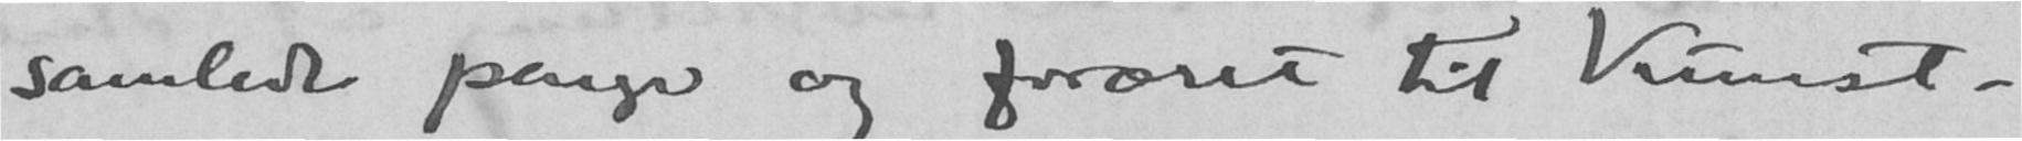samlede penge og foræret til kunst-
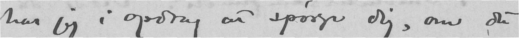har jeg i opdrag at spørge dig, om du
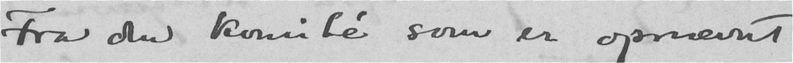Fra den komité som er opnevnt
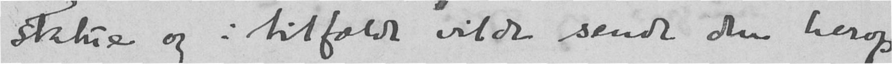statue og i tilfelde vilde sende den herop
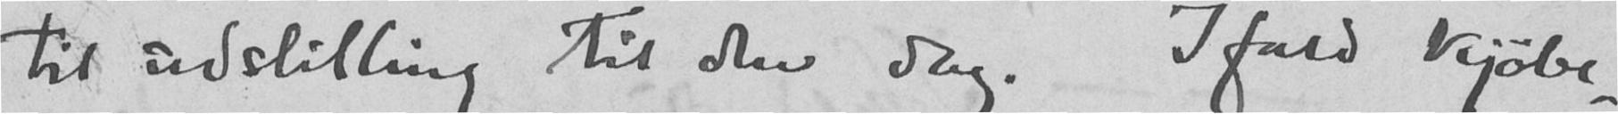til udstilling til den dag. Ifald kjøbe-
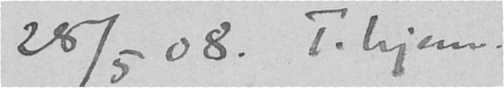28/5 08. T.hjem.
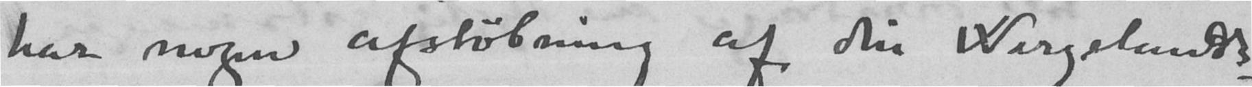har nogen afstøbning af din Wergelands-
